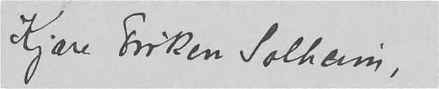Kjære Frøken Solheim,
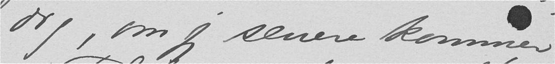dig, om jeg senere kommer
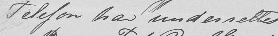Telefon har underrettet
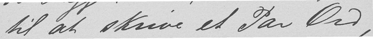til at skrive et Par Ord,
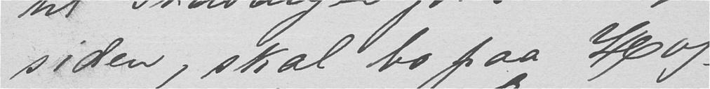siden, skal bo paa Hog-
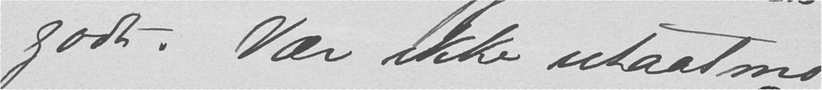godt. Vær ikke utaalmo-
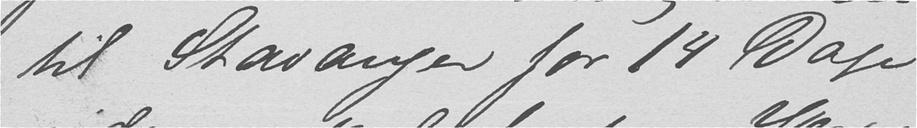til Stavanger for 14 Dage
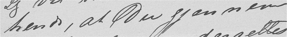hende, at Du gjennem
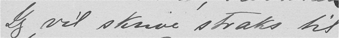Jeg vil skrive straks til
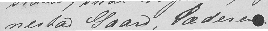nestad Gaard, Sædere
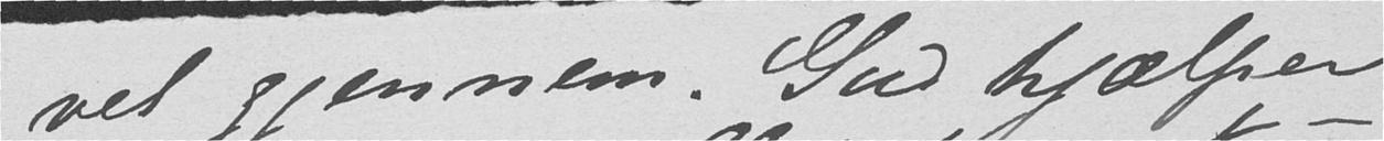vel gjennem. Gud hjælper
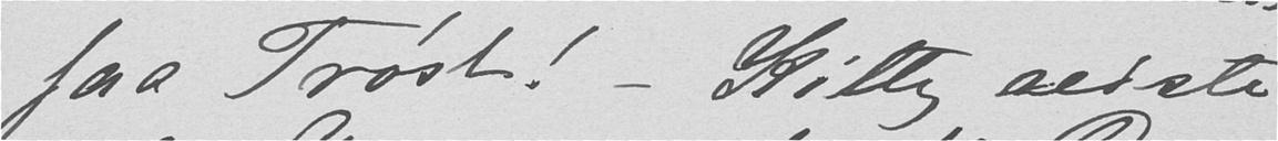faa Trøst! - Kitty reiste
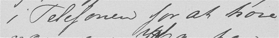i Telefonen for at høre
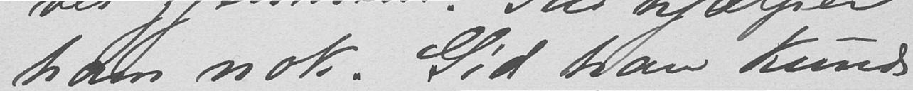ham nok. Gid han kunde
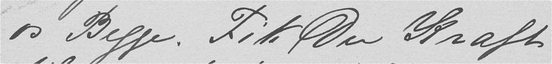os Begge. Fik Du Kraft
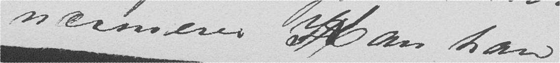nærmere. Kan han
